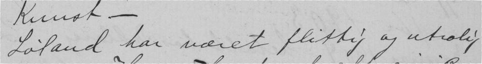Løland har været flittig og utrolig
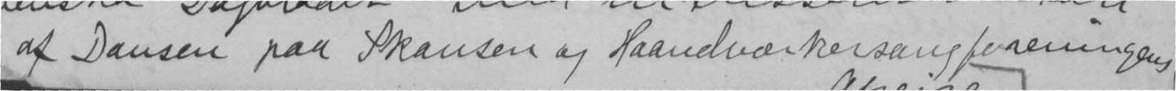af Dansen paa Skansen og Haandværkersangforeningens
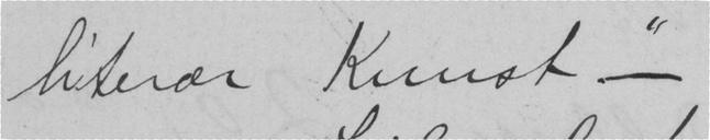literær Kunst" -
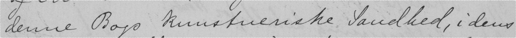denne Bogs kunstneriske Sandhed, i dens
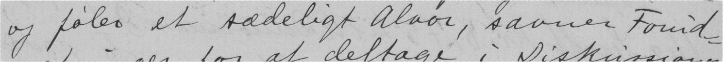og føler et sædeligt Alvor, savner Forud-
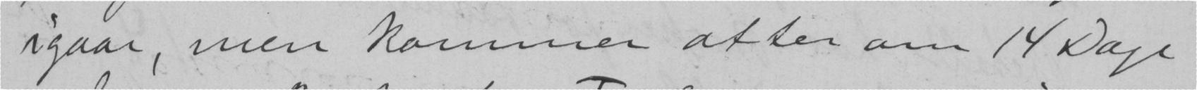igaar, men kommer atter om 14 Dage
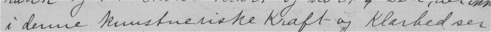i denne kunstneriske Kraft og Klarhed ser
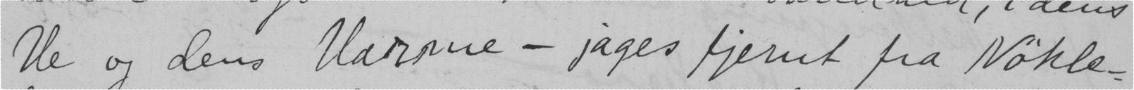Ve og dens Varme - jages fjernt fra Nøkle-
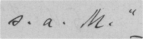s. a. M."
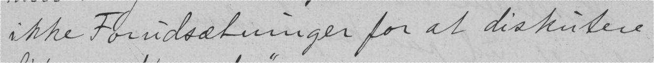ikke Forudsætninger for at diskutere
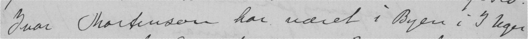Ivar Mortenson har været i Byen i 3 Uger
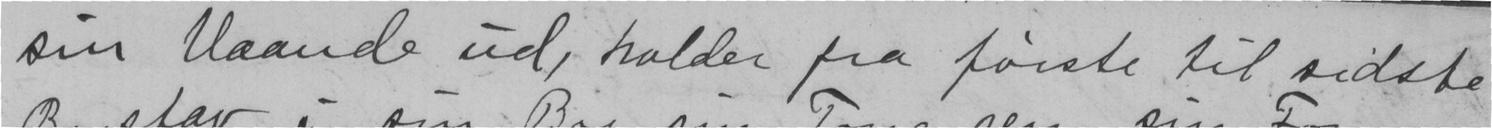sin Vaande ud, holder fra første til sidste
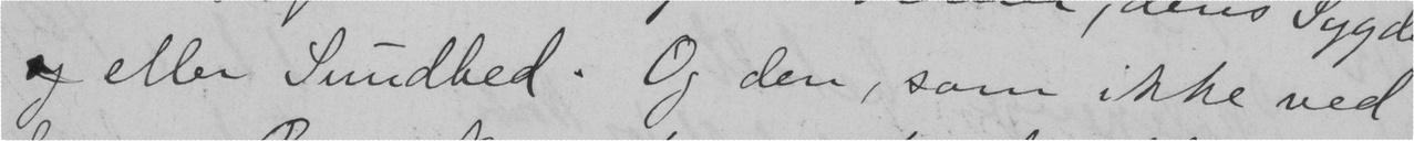og eller Sundhed. Og den, som ikke ved
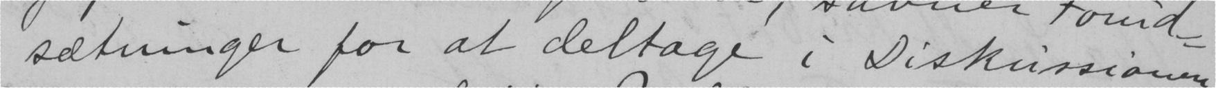sætninger for at deltage i Diskussionen
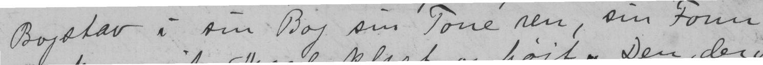Bogstav i sin Bog sin Tone ren, sin Form
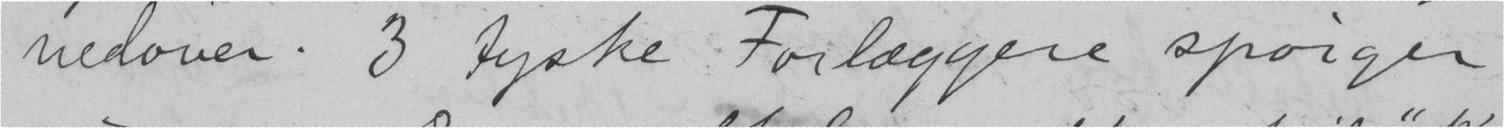nedover. I tyske Forlæggere spørger
